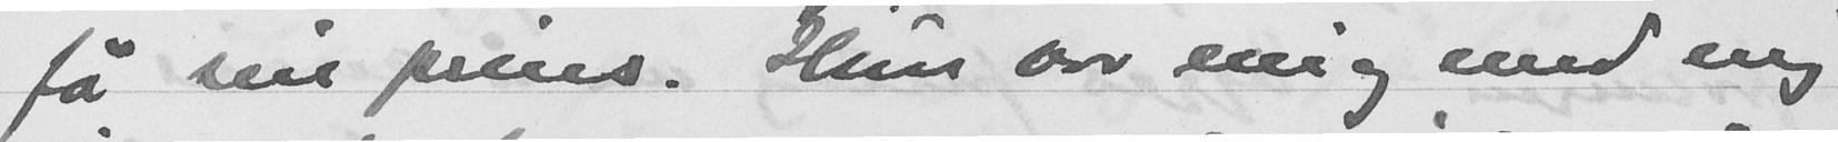få sin prins. Hun var enig med mig
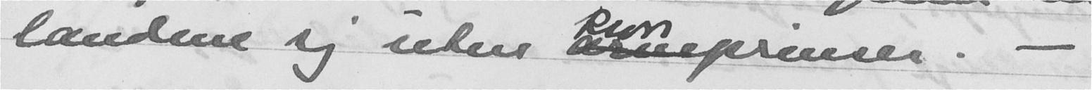landene sig uten kronprinsen. -
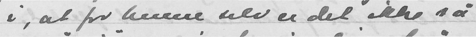i, at for henne selv er det ikke så
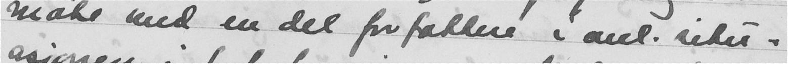møte med en del forfattere i anl. situ-
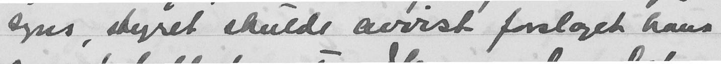syns, styret skulde avvist forslaget hans
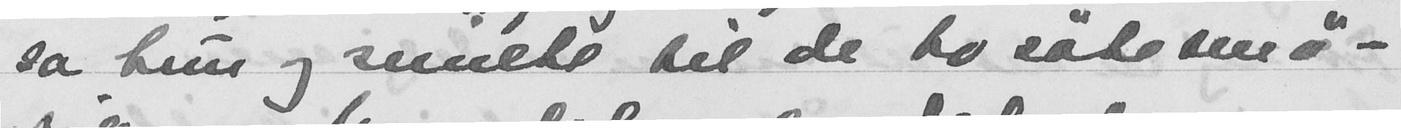sa hun og smilte til de to søte små-
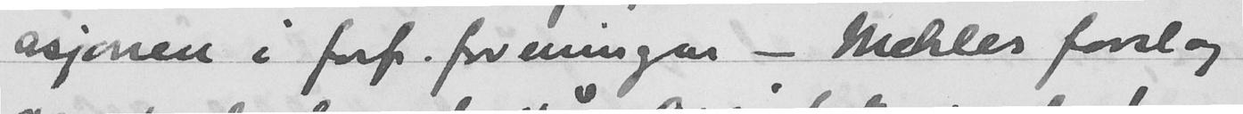asjonen i forf. foreningen - Mæhles forslag
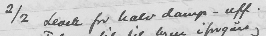2/2 Lever for halv damp - uff.
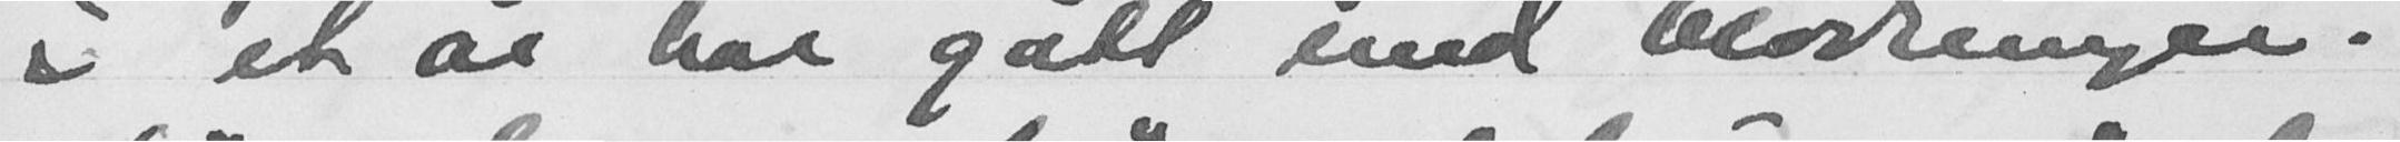i et år har gått med blødningen.
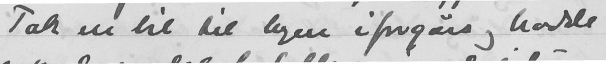Tok en bil til byen iforgårs og hadde
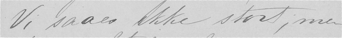Vi saaes ikke stort, men
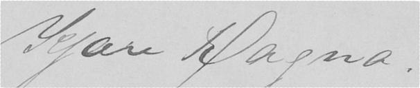Kjære Ragna.
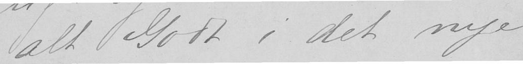alt Godt i det nye
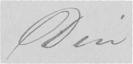Din
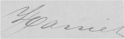Harriet
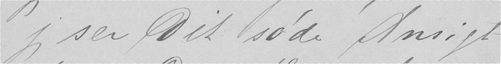jeg ser Dit søde Ansigt.
